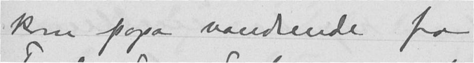kom papa vandrende fra
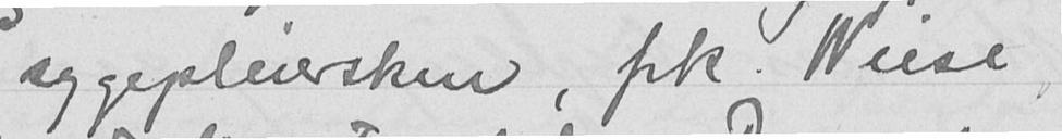sygepleiersken, frk. Wiese,
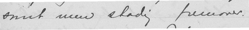somt men stadig fremover.
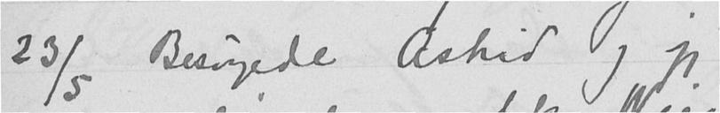23/5. Besøgede Astrid og jeg
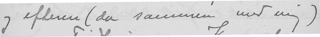og efterm (da sammen med mig)
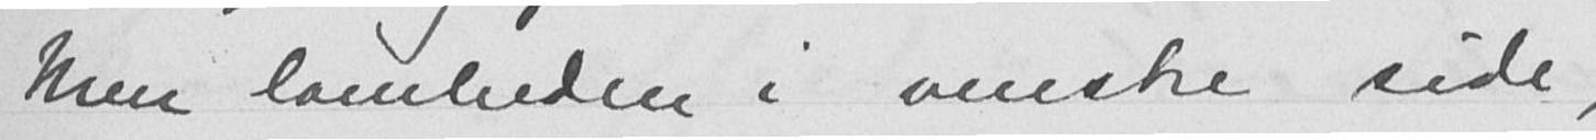Men lamheden i venstre side,
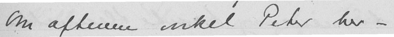Om aftenen onkel Peter her -
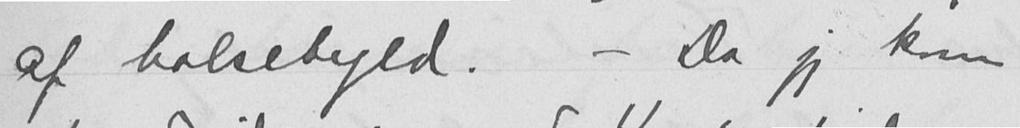af halsebyld. - Da jeg kom
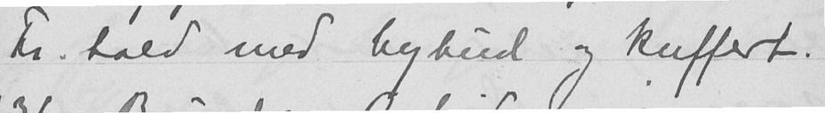Fr. hald med bybud og kuffert.
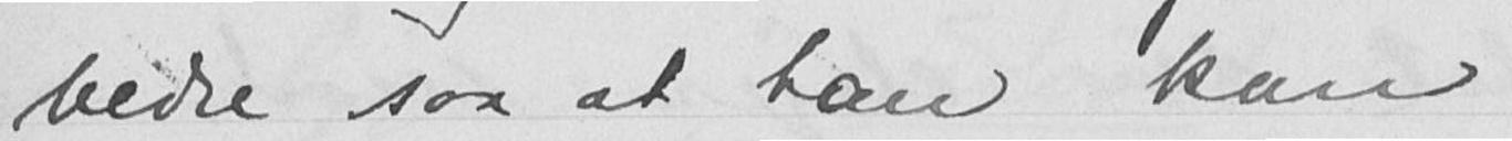bedre saa at han kan
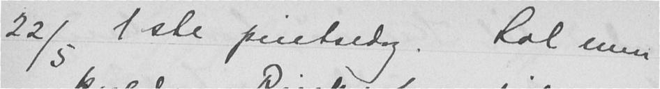22/5 1ste pintsedag. Sol men
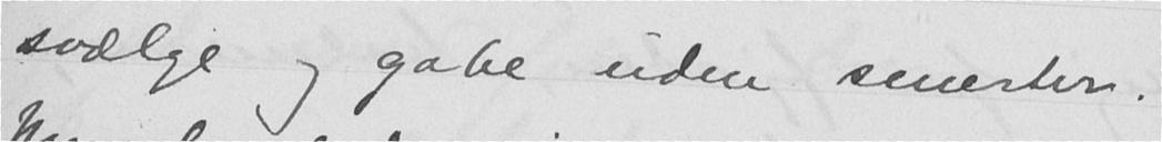svælge og gabe uden smerter.
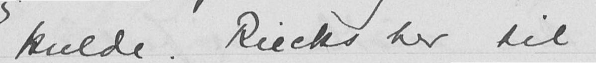kulde. Riecks her til
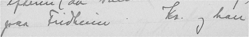paa Fridheim. Kr. og han
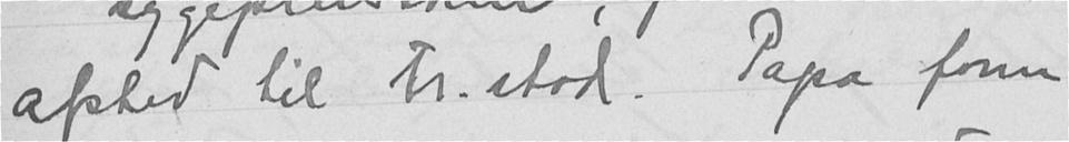afsted til Fr. stad. Papa form
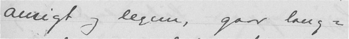ansigt og legem, gaar lang-
